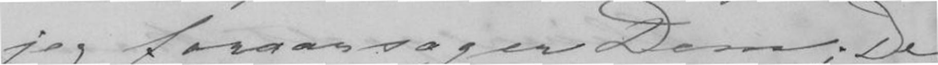jeg foraarsager Dem, De
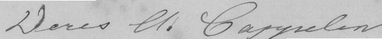Deres M. Cappelen
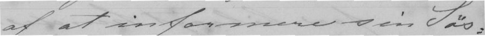af at informere sin Søs-
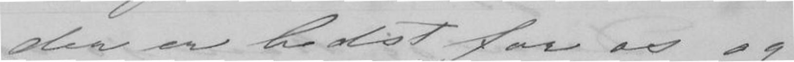der er bedst for os og
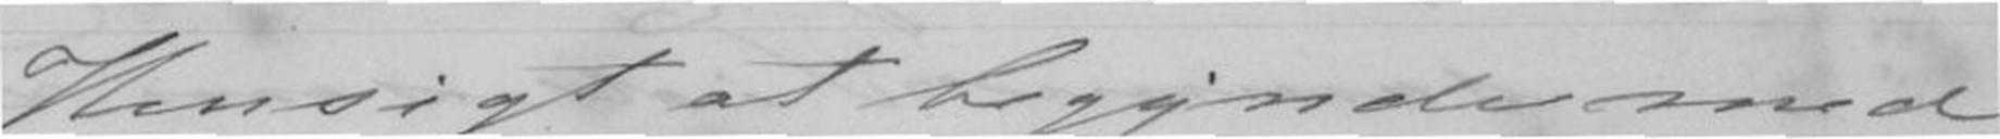Hensigt at begynde med
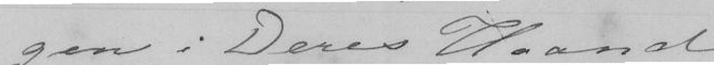gen i Deres Haand
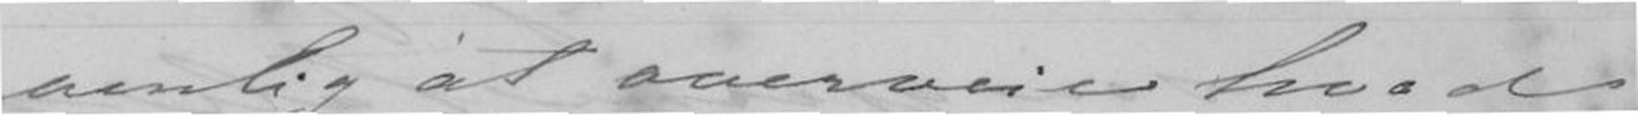venlig at overveie hvad
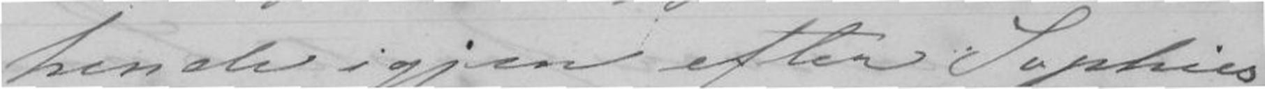hende igjen efter Sophies
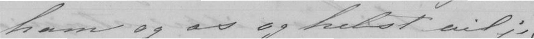ham og os og helst vil jeg
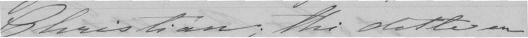til Christian, thi dette er
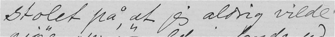stolet på, at jeg aldrig vilde
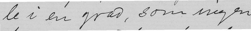le i en grad, som ingen
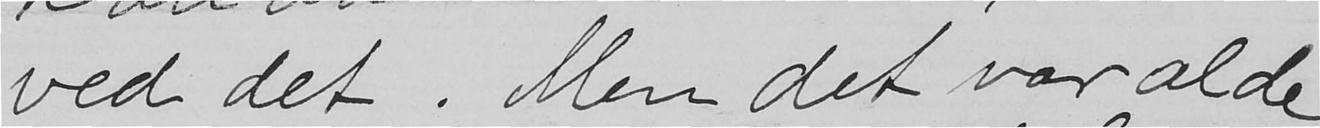ved det. Men det var alde
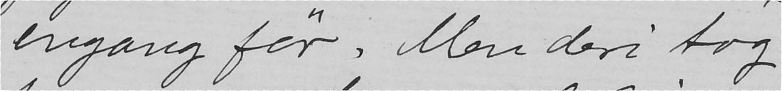engang før. Men deri tog
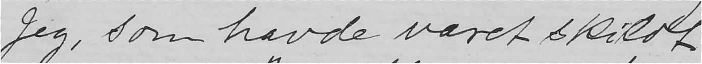Jeg, som havde været skildt
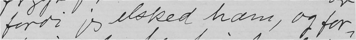fordi jeg elsked ham, og for-
